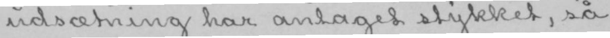udsætning har antaget stykket, så
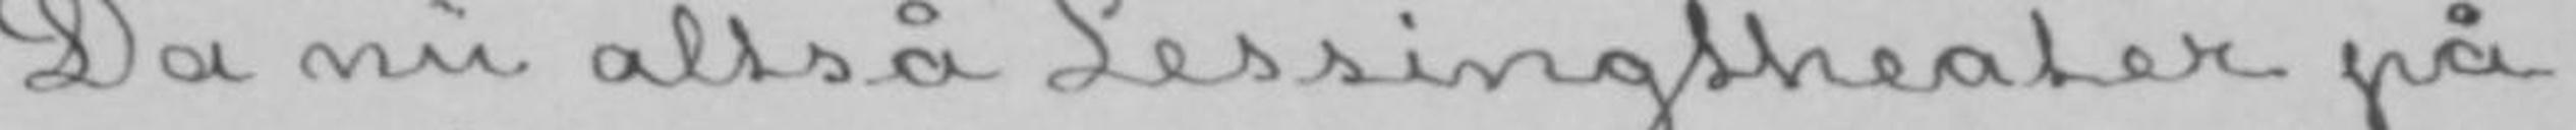Da nu altså Lessingtheater på
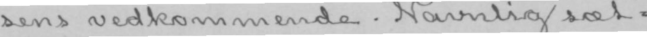sens vedkommende. Navnlig sæt-
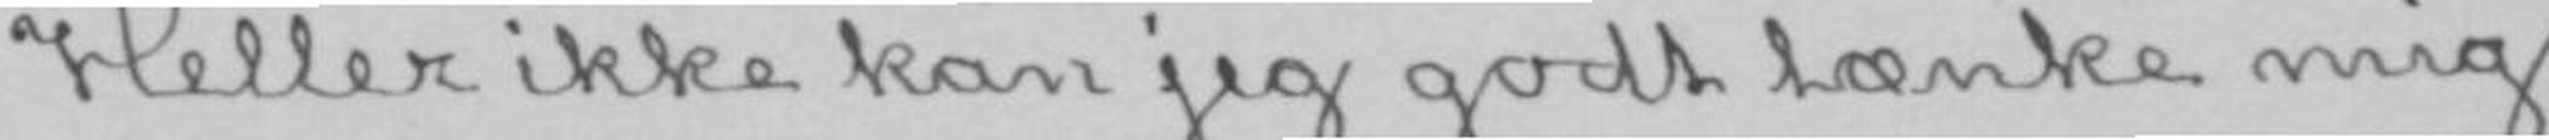Heller ikke kan jeg godt tænke mig
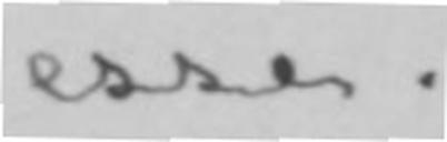esse.
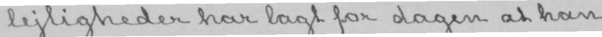lejligheder har lagt for dagen at han
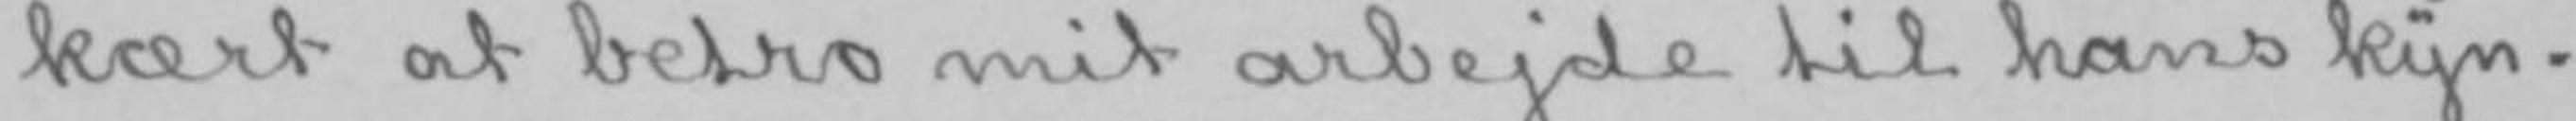kært at betro mit arbejde til hans kyn-
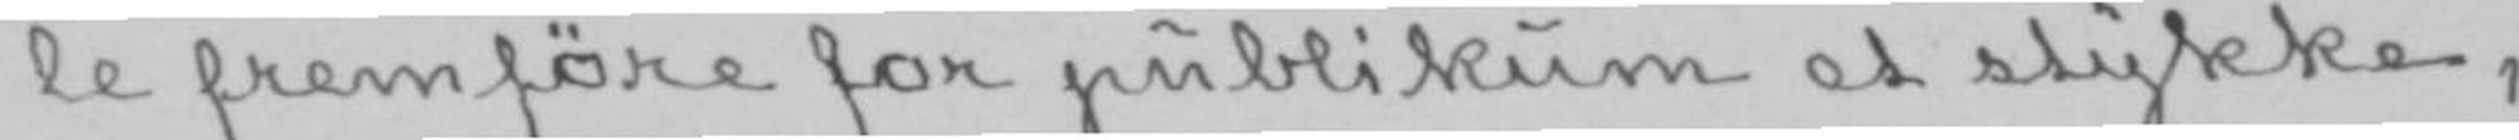le fremføre for publikum et stykke,
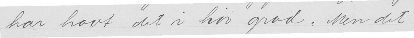har havt det i høi grad. Men det
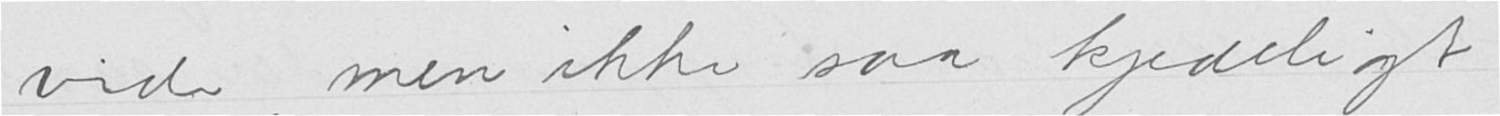vide, men ikke saa kjedeligt
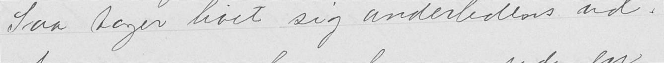Saa tager livet sig anderledes ud.
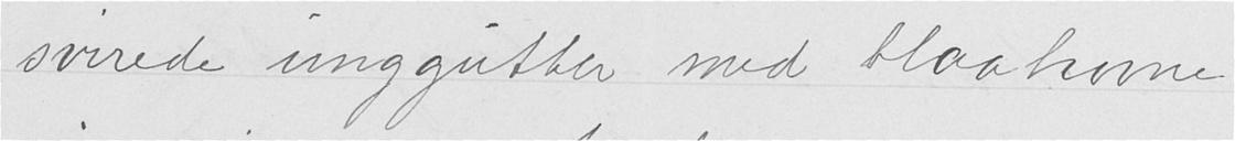svirede unggutter med blaahovne
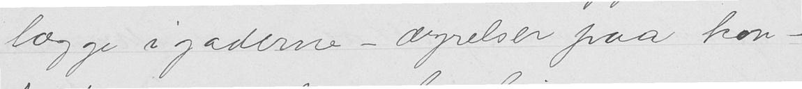lægge i gaderne - ærgrelser paa kon-
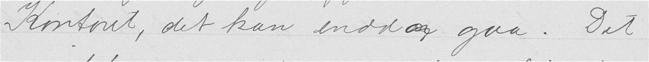Kontoret, det kan endda gaa. Det
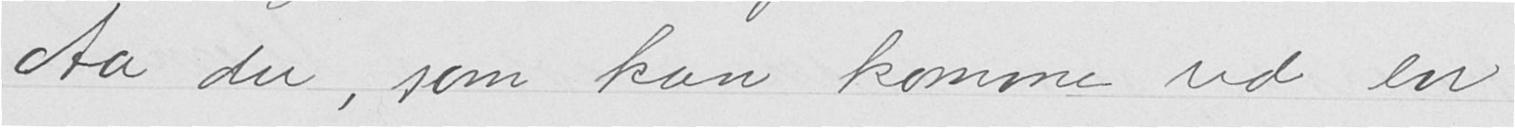Aa du, som kan komme ud en
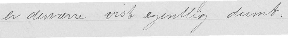er desværre vist egentlig dumt.
